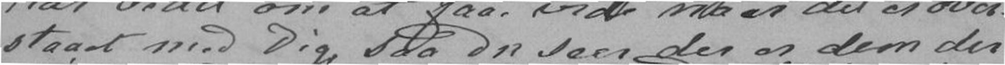staaer med Dig, saa Du seer der er dem der
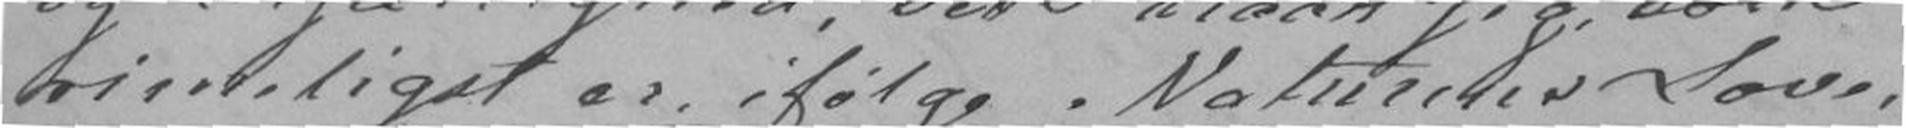rimeligst er ifølge Naturens Love,
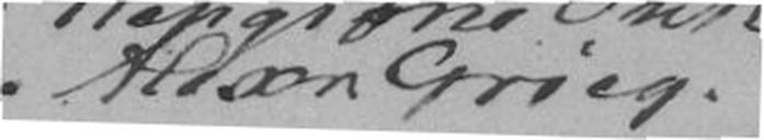Alexn Grieg.
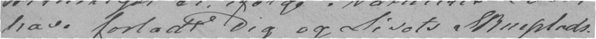have forladt Dig og Livets Skueplads.
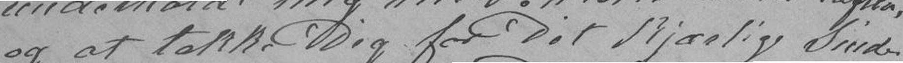og at takke Dig for Dit kjærlige Sinde-
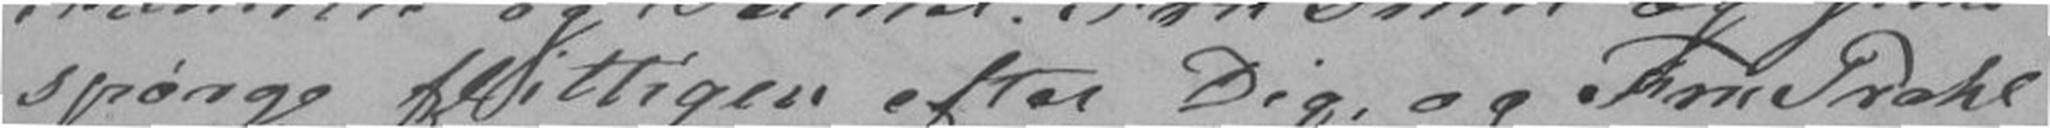spørge flittigen efter Dig og Fru Prahl
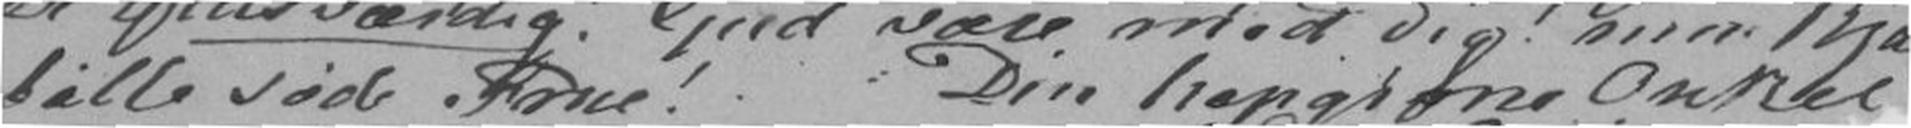lille søde Frue!Din hengivne Onkel
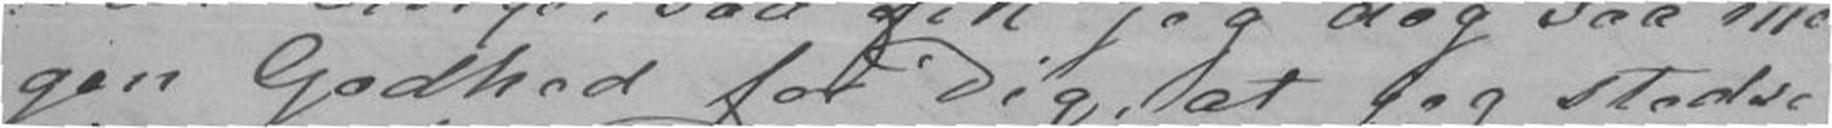gen Godhed for Dig, at jeg stedse
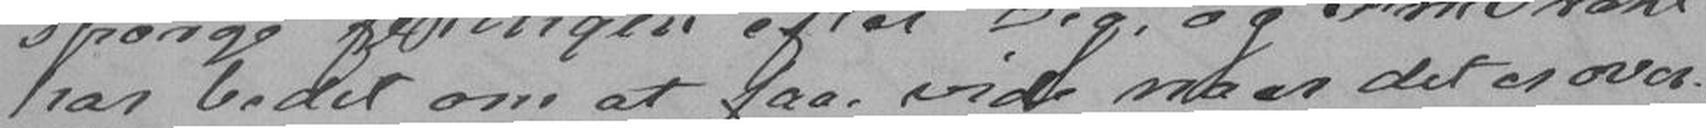har bedet om at faae vide naar det er over-
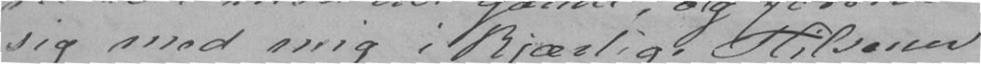sig med mig i Kjærlige Hilsener
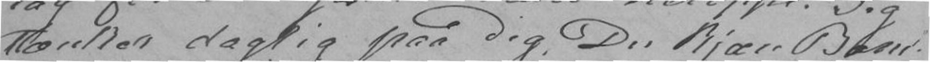tænker daglig paa Dig, Du kjære Barn
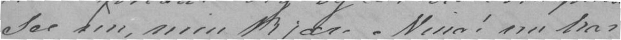See nu, min kjære Nina nu har
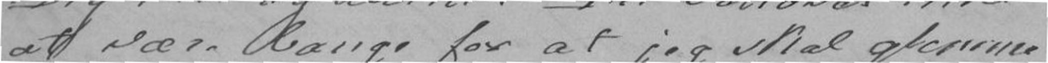at være bange for at jeg skal glemme
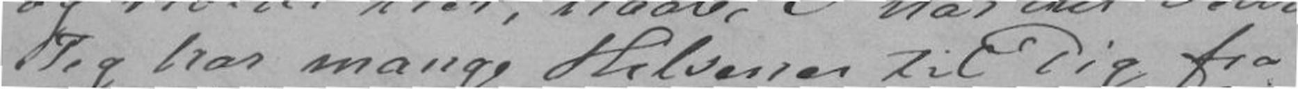Jeg har mange Hilsener til Dig fra
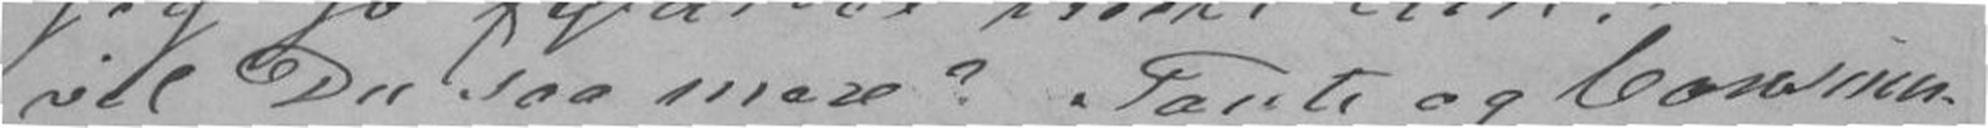vil Du saa mere? Tante og Cousiner-
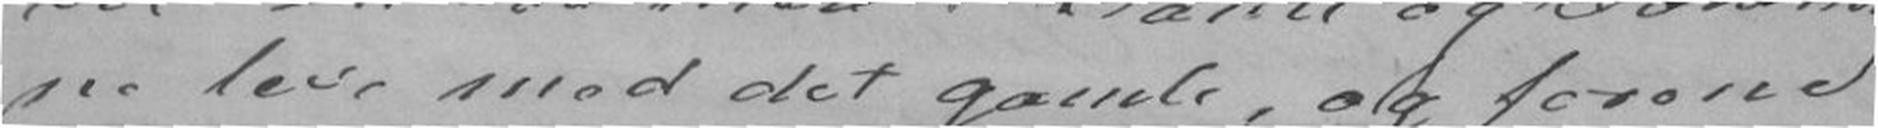ne leve med det gamle, og forene
